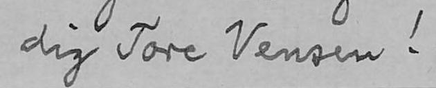dig Tore Vensen!
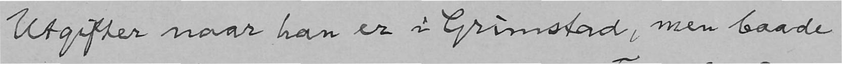Utgifter naar han er i Grimstad, men baade
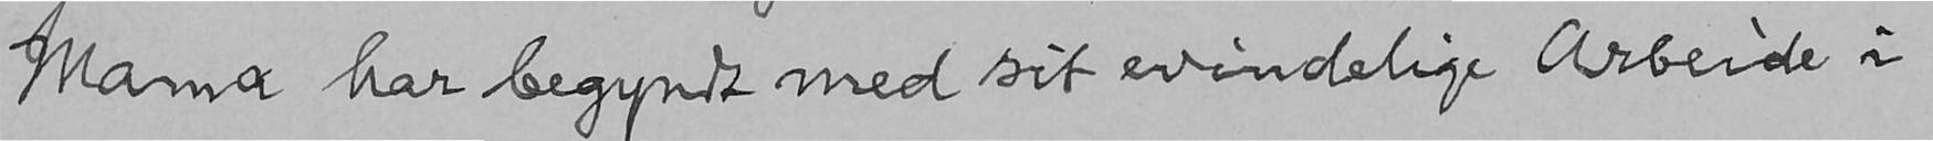Mama har begyndt med sit evindelige Arbeide i
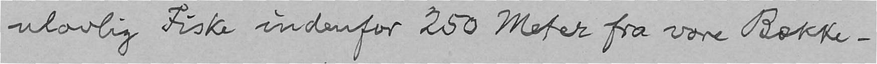ulovlig Fiske indenfor 250 Meter fra vore Bække-
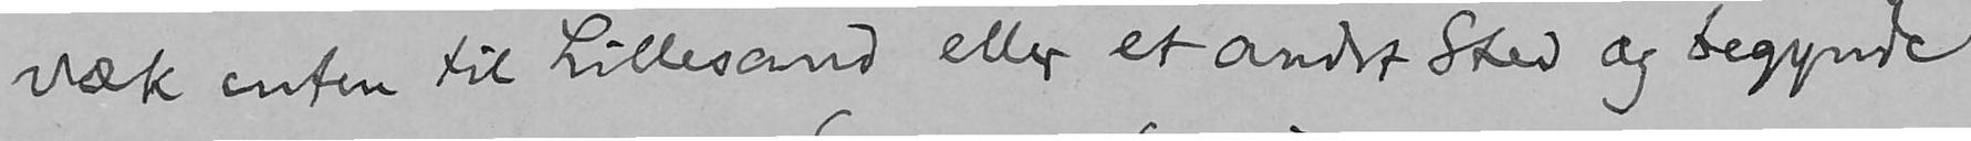væk enten til Lillesand eller et andet Sted og begynde
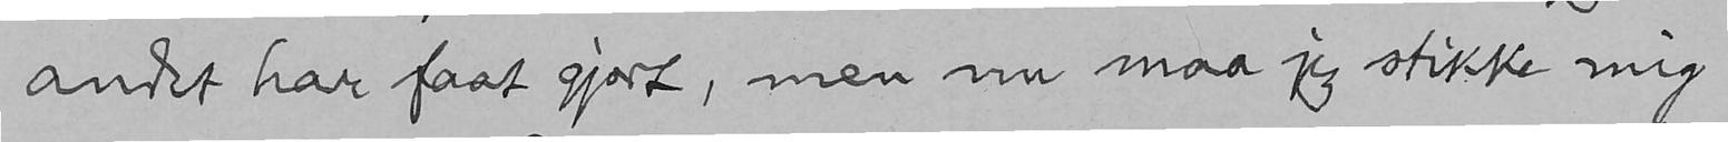andet har faat gjort, men nu maa jeg stikke mig
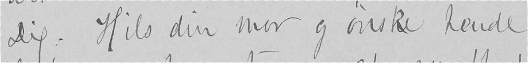Dig. Hils din mor og ønske hende
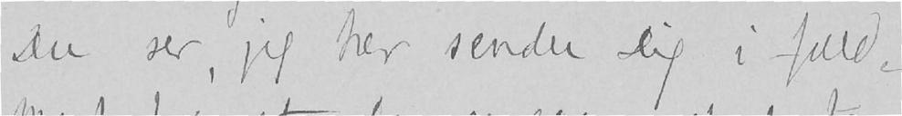Du ser, jeg her sender Dig i fuld-
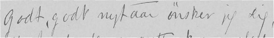Godt, godt nytaar ønsker jeg Dig,
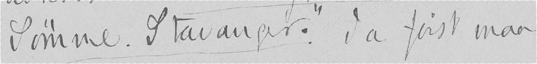Sømme. Stavanger.» Ja først maa
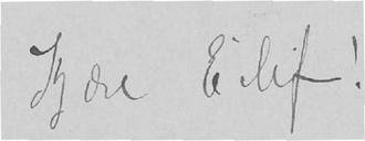Kjære Eilif!
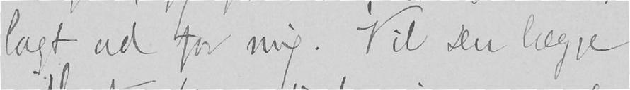lagt ud for mig. Vil Du lægge
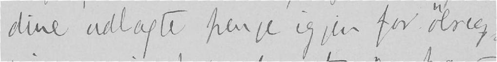dine udlagte penge igjen for ølreg-
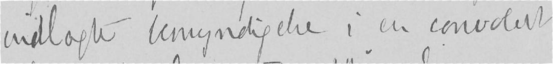indlagte bemyndigelse i en convolut
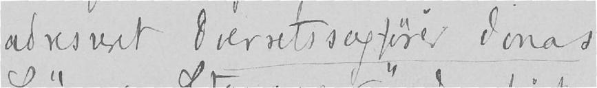adresseret Overretssagfører Jonas
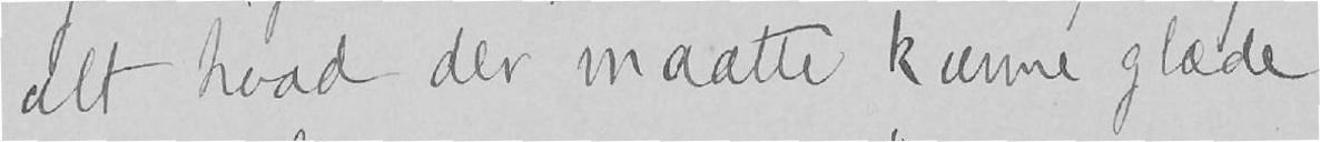alt hvad der maatte kunne glæde
